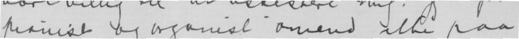pianist og organist omend ikke paa
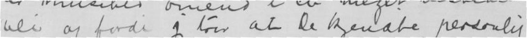vei og fordi jeg tror at De kjendte personlig
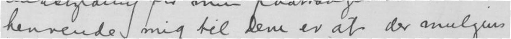henvende mig til Dem er at der muligen
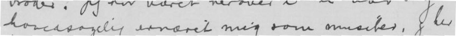hovedsagelig ernæret mig som musiker, og har
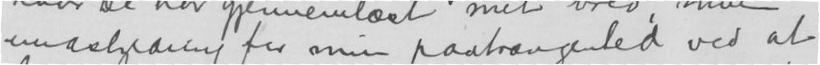undskylding fra min paatrænghed ved at
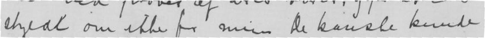skyld om ikke for min De kanske kunde
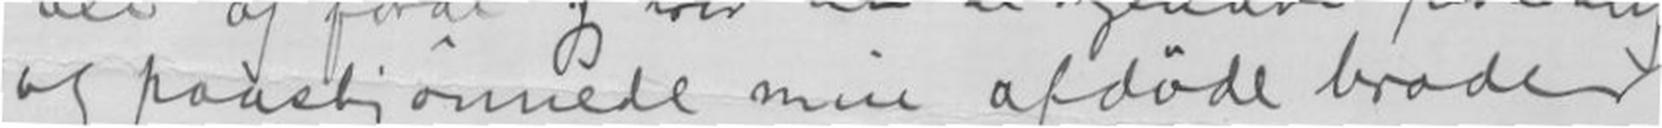og paaskjønnede min afdøde broder
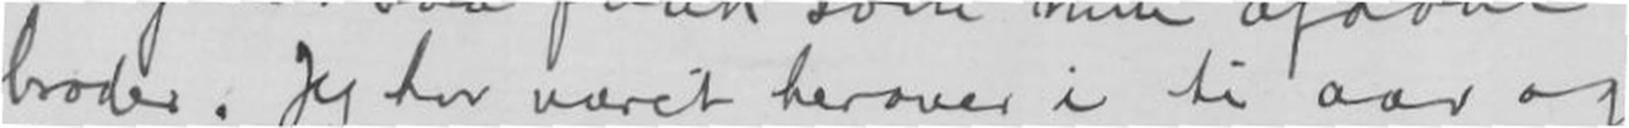broder. Jeg har været herover i ti aar og
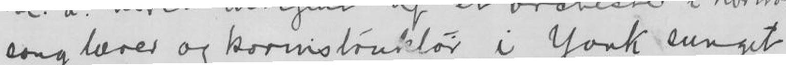sanglærer og korinstrutør i York sunget
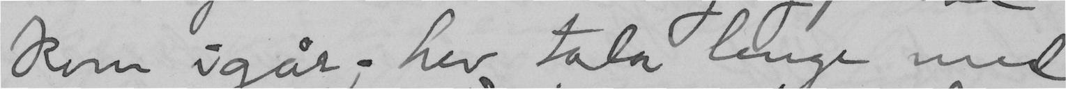kom igår; hev tala længe med
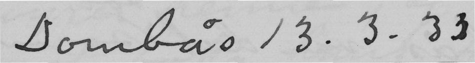Dombås 13.3.33
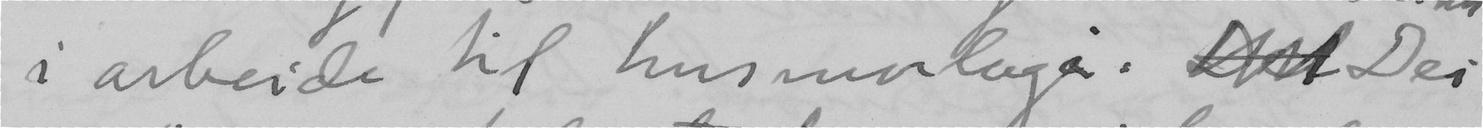i arbeide til husmorlage. Dei
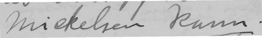Mickelsen kann.
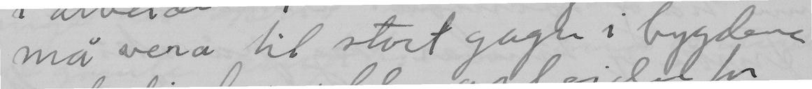må vera til stort gagn i bygdene
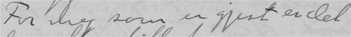For meg som er gjest er det
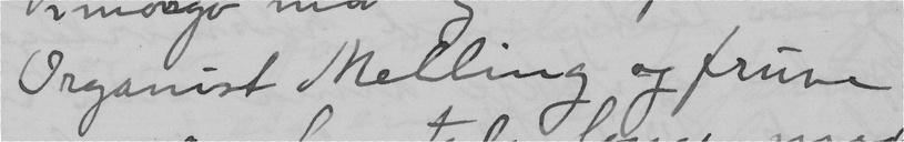Organist Melling og frue
